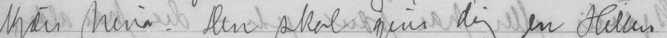kjære Nina. Den skal give Dig en Hilsen
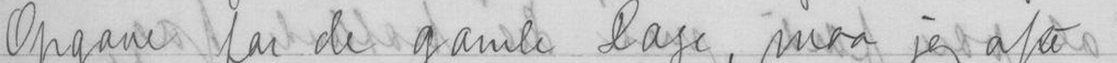Opgave for de gamle Dage, maa jeg ofte
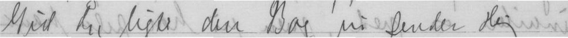Gid du ligte den Bog vi sender Dig
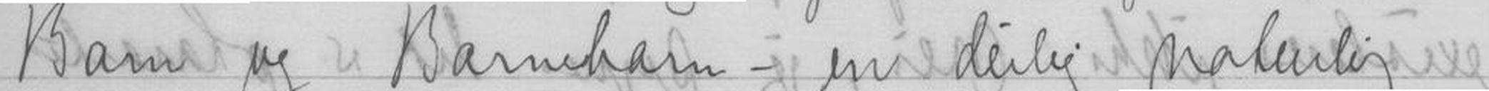Barn og Barnebarn - en deilig naturlig
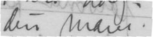Din Marie
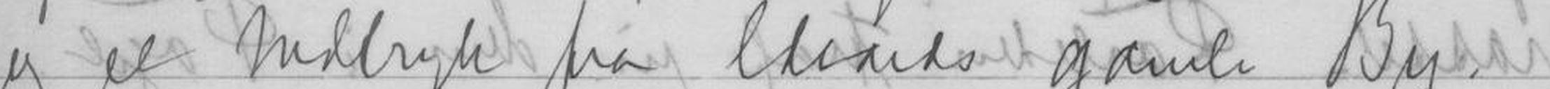og et Indtryk fra Edvards gamle By.
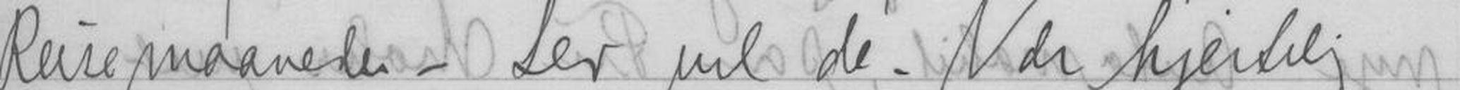Reisemaaneder. - Lev vel da. Vær hjertelig
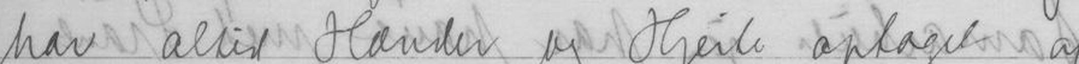har altid Hænder og Hjerte optaget af
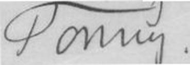Tonny.
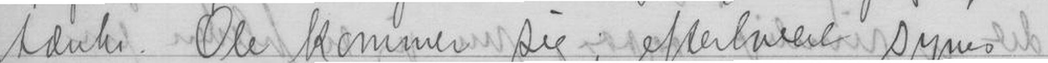tænke. Ole kommer sig, efterhvert synes
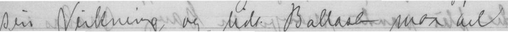sin Virkning og lidt Ballast maa vel
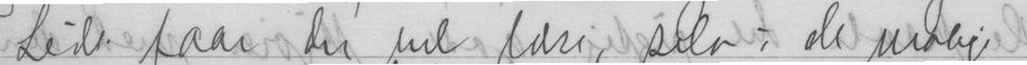Lidt faar du vel læst, selv i de urolige
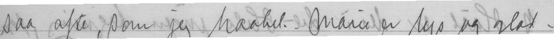saa ofte, som jeg haabet. Marie er lys og glad -
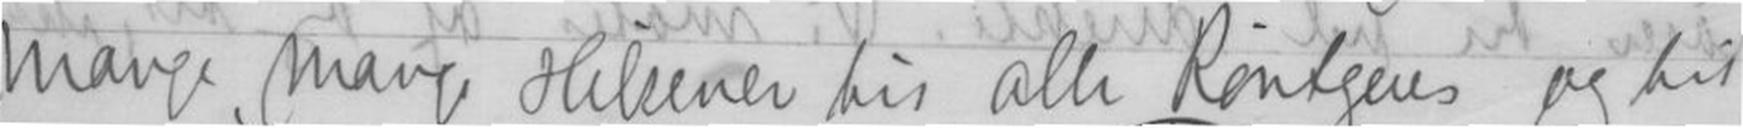Mange, mange Hilsener til alle Röntgens og til
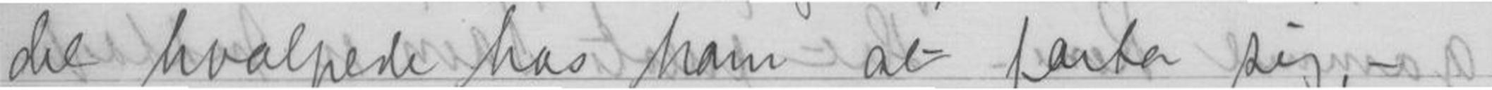det hvalpede hos ham at forta sig, -
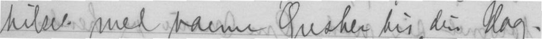hilset med varme Ønsker til Din dag
